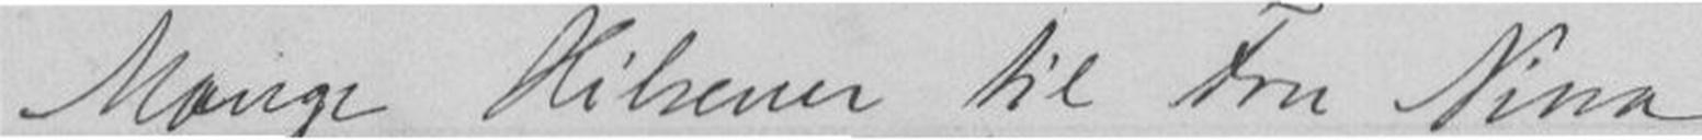Mange Hilsener til Fru Nina
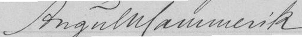Angul Hammerich
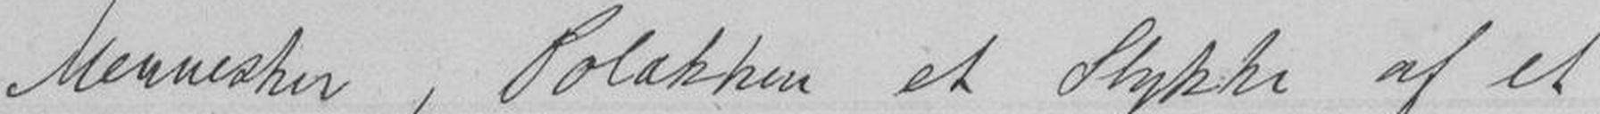Mennesker, Polakken et stykke af et
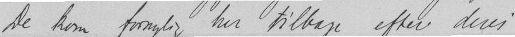De kom fornylig her tilbage efter deres
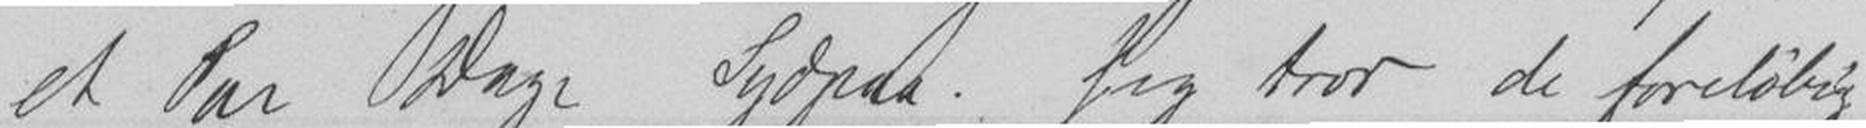et Par Dage Sydpaa. Jeg tror de foreløbig
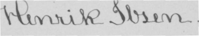Henrik Ibsen.
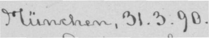München, 31.3.90.
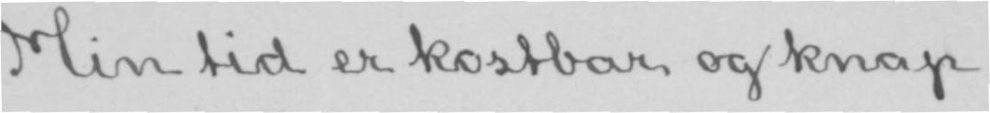Min tid er kostbar og knap.
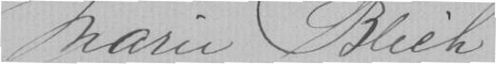Marie Blich
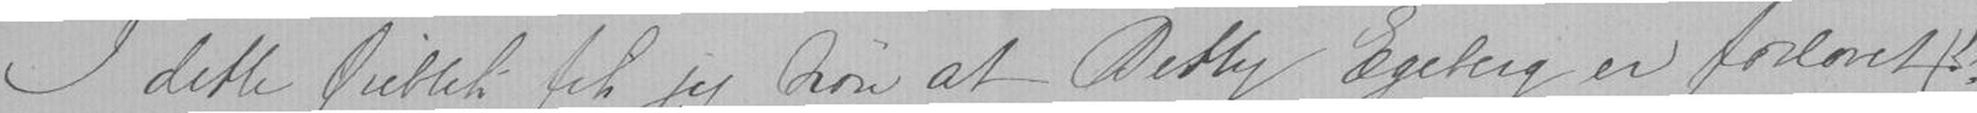I dette Øieblik fik jeg høre at Betty Egeberg er forlovet(!!)
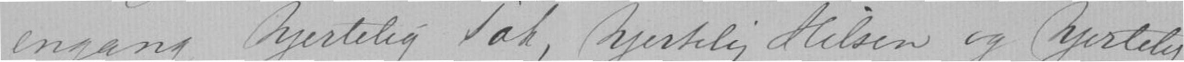engang hjertelig Tak, hjertelig Hilsen og hjertelig
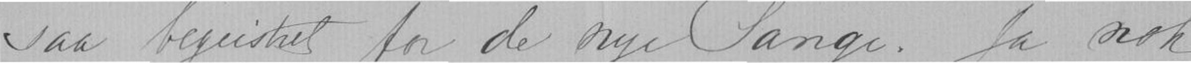saa begeistret for de nye Sange. Ja nok
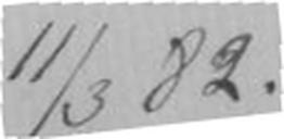11/3 82
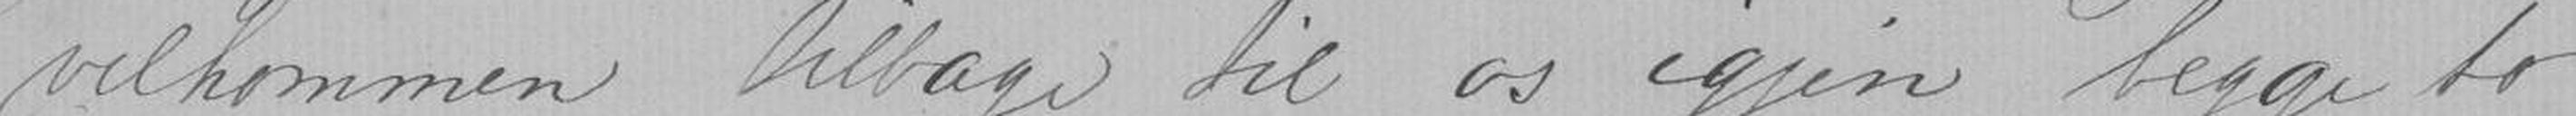velkommen tilbage til os igjen begge to!
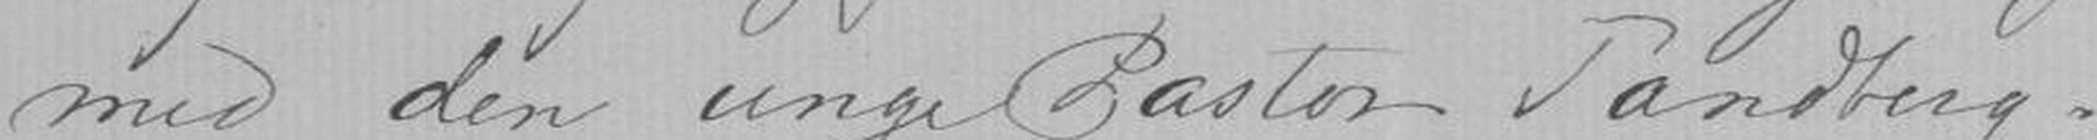med den unge Pastor Tanberg.
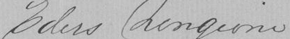Eders hengivne
